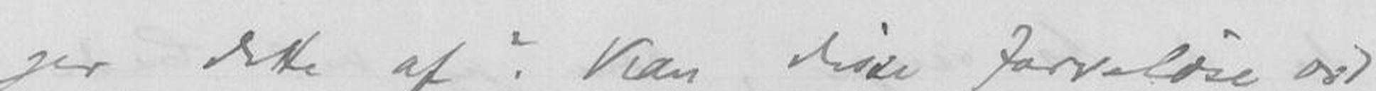ger dette af? Kan disse farveløse ord
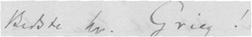Bedste hr. Grieg!
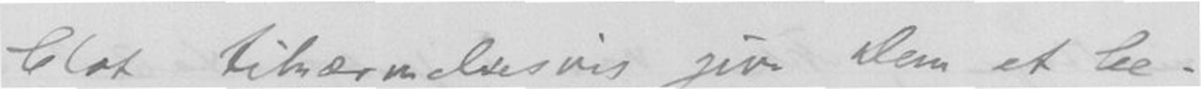blot tilnærmelsesvis give Dem et be-
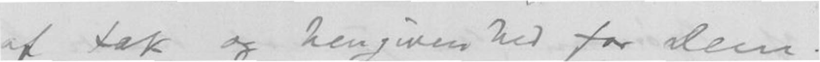af tak og hengivenhed for Dem.
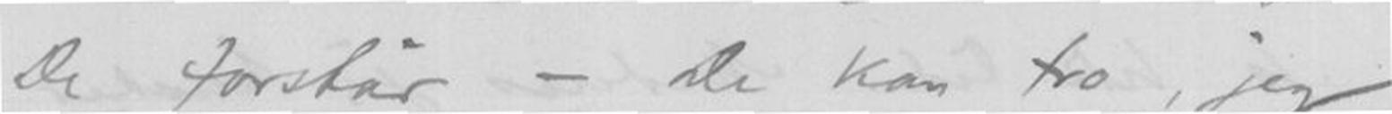De forstår - De kan tro, jeg
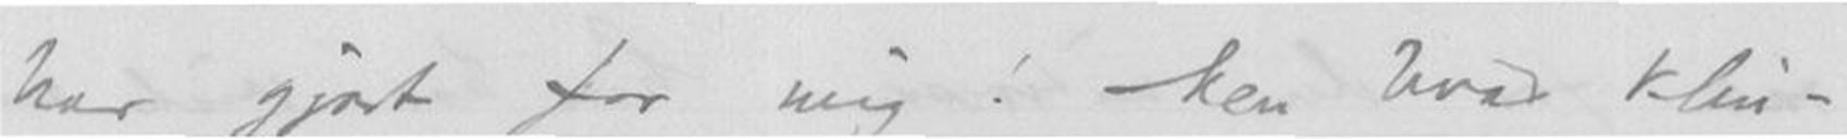har gjort for mig! Men hvad klin-
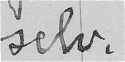selv.
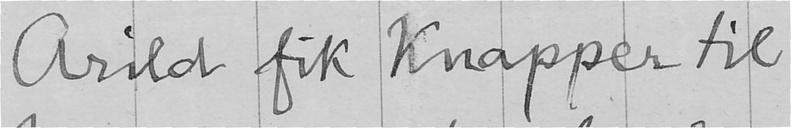Arild fik Knapper til
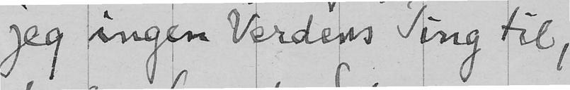jeg ingen Verdens Ting til,
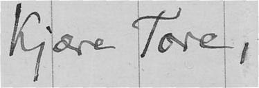Kjære Tore,
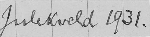Julekveld 1931.
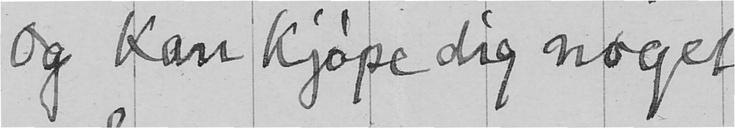og kan kjøpe dig noget
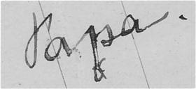Papa.
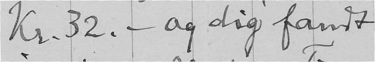Kr. 32. - og dig fandt
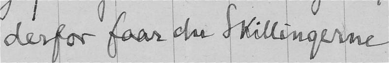derfor faar du Skillingerne
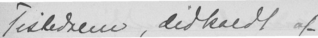Tistedalen, didkaldt af
